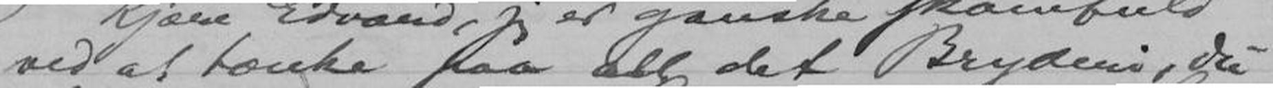ved at tænke paa alt det Bryderi, Du
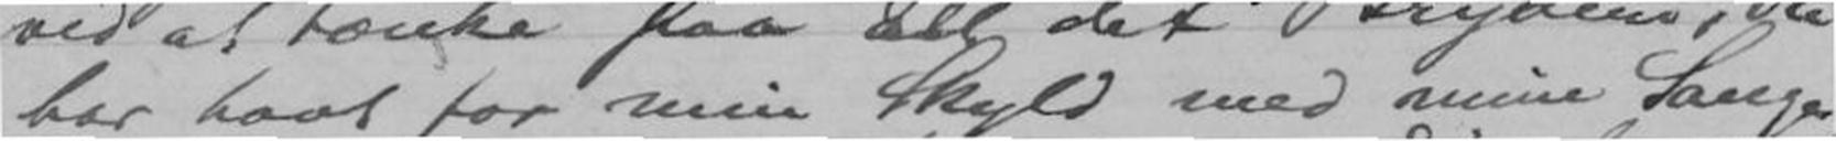har havt for min Skyld med mine Sange!
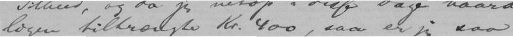ligen tiltrængte Kr. 400, saa er jeg saa
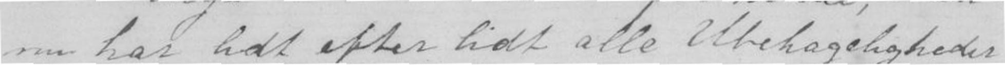nu har lidt efter lidt alle Ubehageligheder
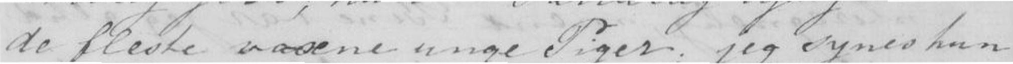de flæste væsene unge Piger; jeg synes hun
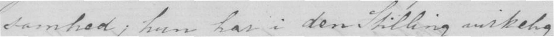somhed, hun har i den Stilling virkelig
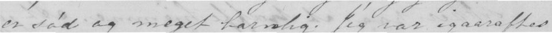er sød og meget barnlig. Jeg var igaaraftes
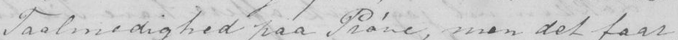Taalmodighed paa Prøve, men det faar
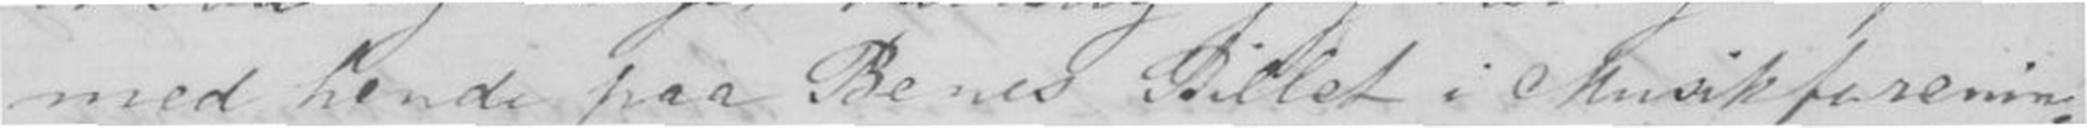med hende paa Benes Billet i Musikforenin-
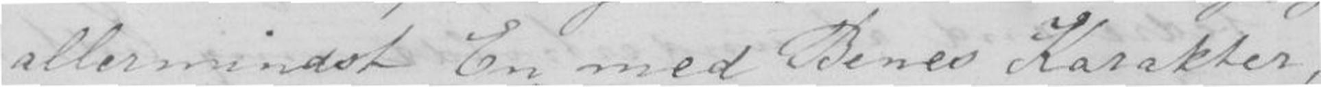allermindst En med Benes Karakter,
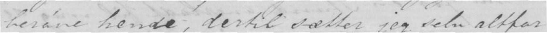berøve hende, dertil sætter jeg selv altfor
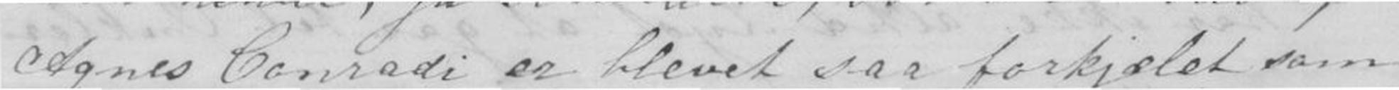Agnes Conradi er bleven saa forkjælet som
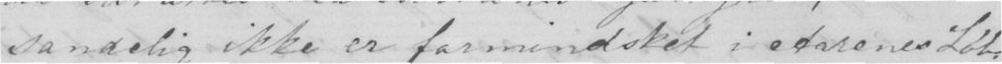sandelig ikke er formisdsket i Aarenes Løb;
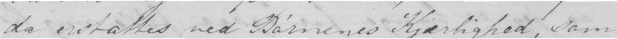da erstattes ved Børnenes Kjærlighed, som
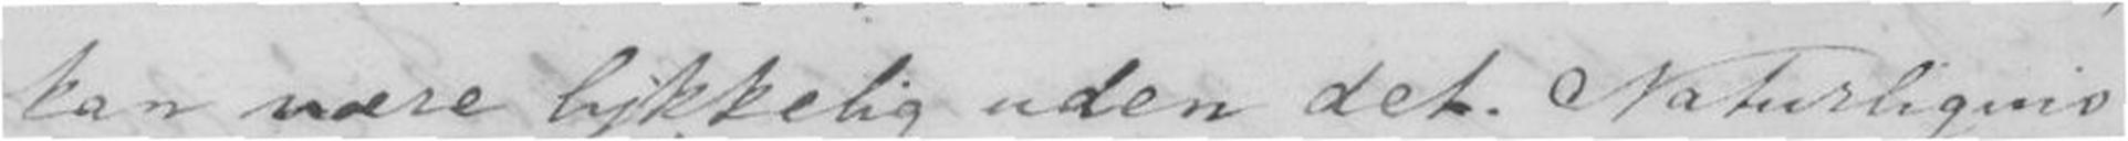kan være lykkelig uden det. Naturligvis
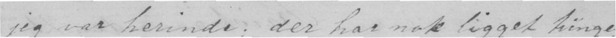jeg var herinde; der har nok ligget tunge,
